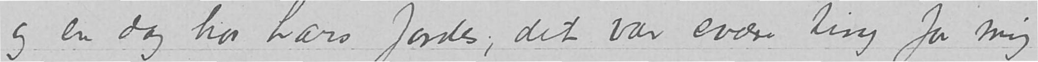og en dag hos Lars Jordes; det var svære ting for mig
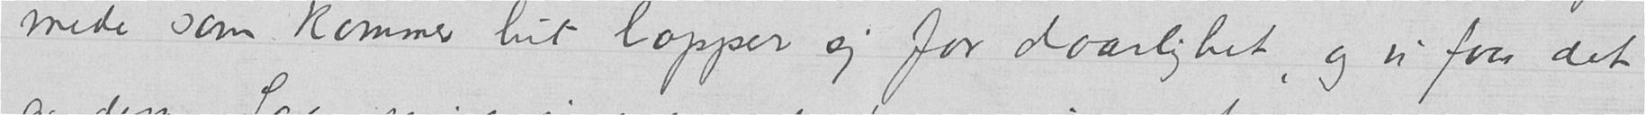mede som kommer hit lopper sig for daarlighet, og vi faar det
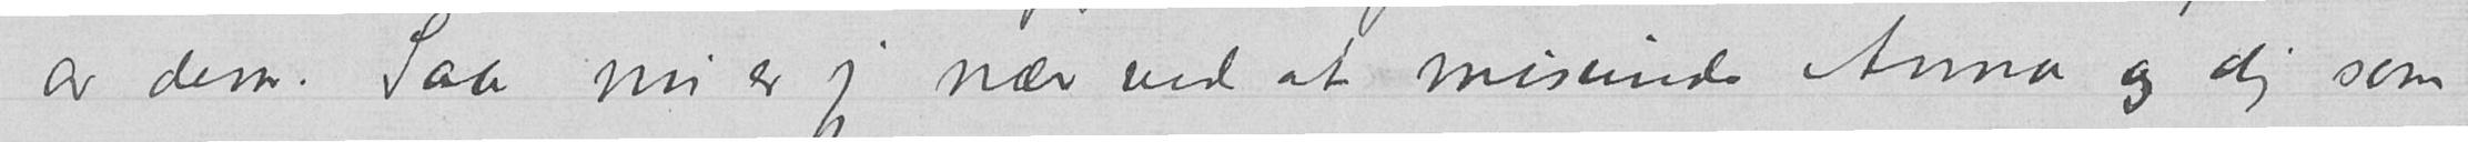av dem. Saa nu er jeg nær ved at misunde Anna og dig som
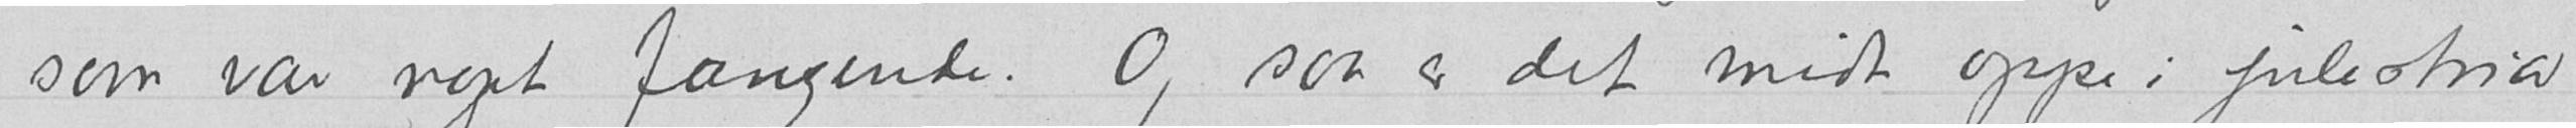som var noget fængende. Og saa er det midt oppe i julestria
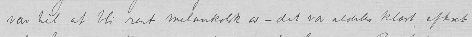var til at bli rent melankolsk av – det var aldeles klart efterat
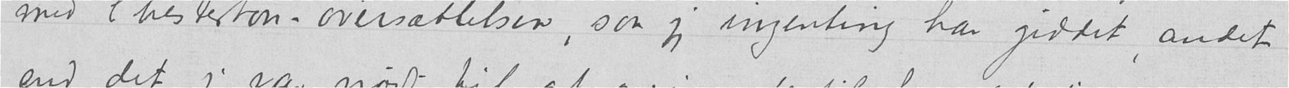med Chesterton-oversættelsen, saa jeg ingenting har giddet, andet
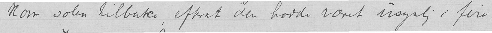kom solen tilbake, efterat den hadde været usynlig i fire
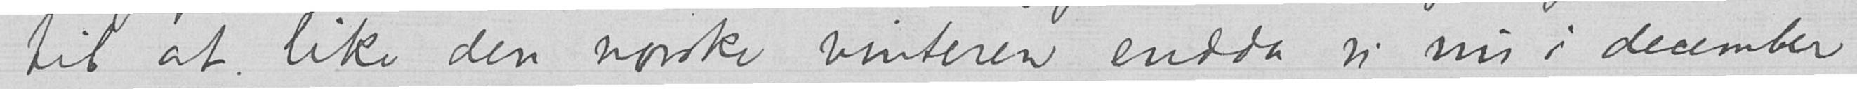til at like den norske vinteren, endda vi nu i december
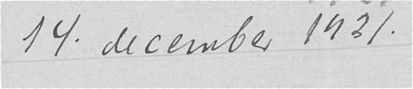14. december 1931.
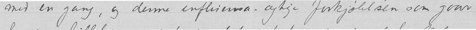med en gang, og denne influensa-agtige forkjølelsen som gaar
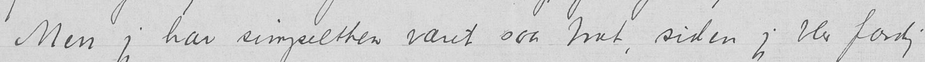Men jeg har simpelthen været saa træt siden jeg blev færdig
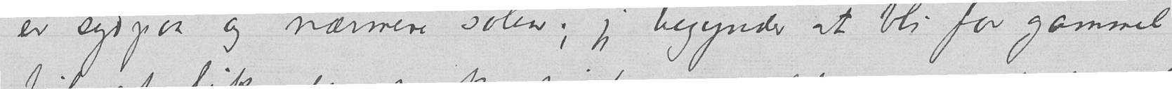er sydpaa og nærmere solen; jeg begynder at bli for gammel
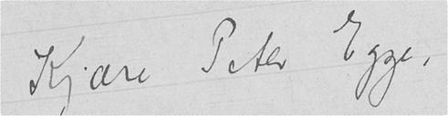Kjære Peter Egge,
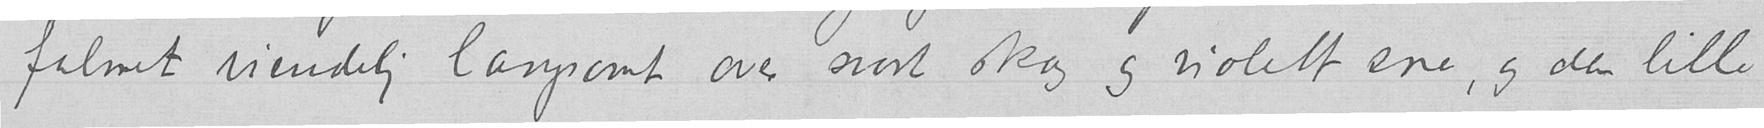falmet uendelig langsomt over svart skog og violett sne, og den lille
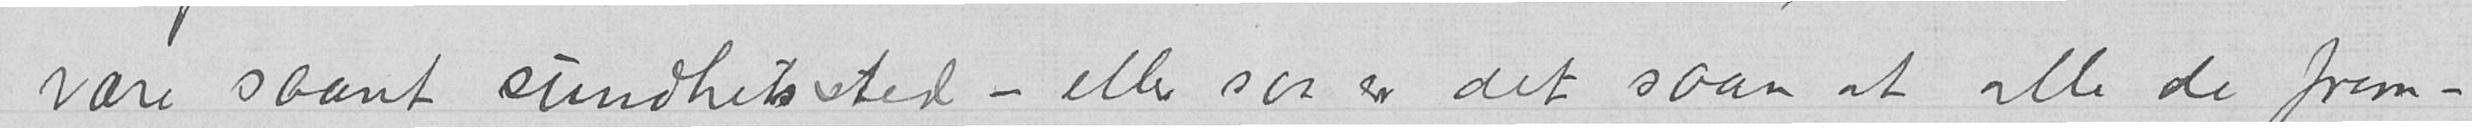være saant sundhetssted - eller saa er det saan at alle de frem-
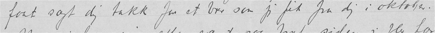faat sagt dig takk for et brev som jeg fik fra dig i oktober.
